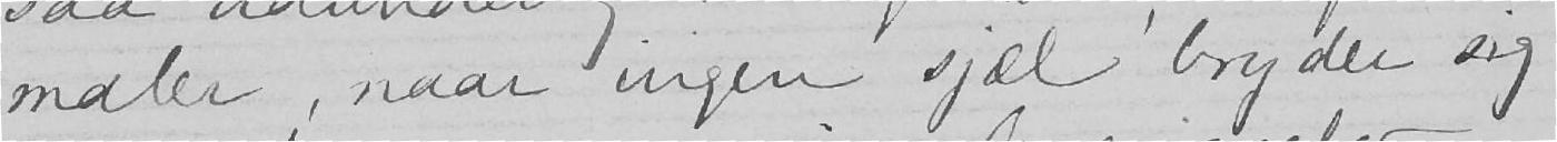maler, naar ingen sjæl bryder sig
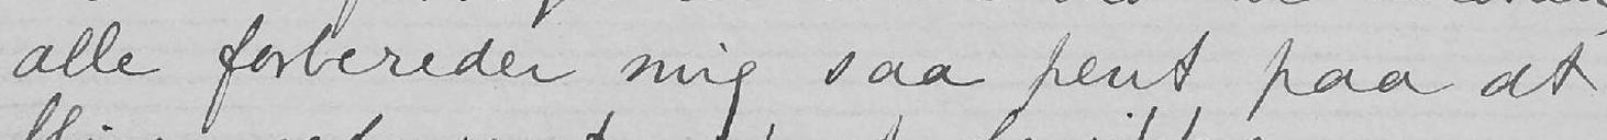alle forbereder mig saa pent paa at
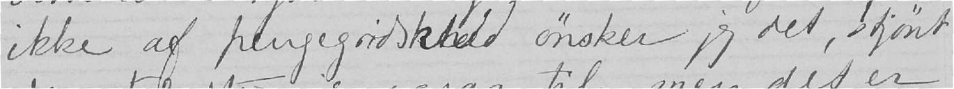ikke af pengegridskhed ønsker jeg det, skjønt
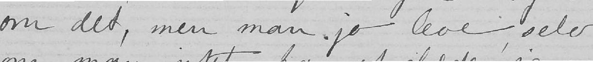om det, men man jo leve, selv
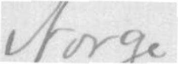Norge
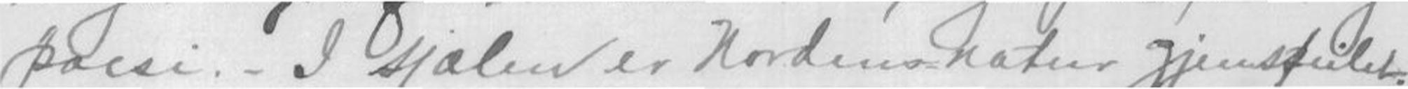poesi. - I sjælen er Nordens=natur gjenspeilet.
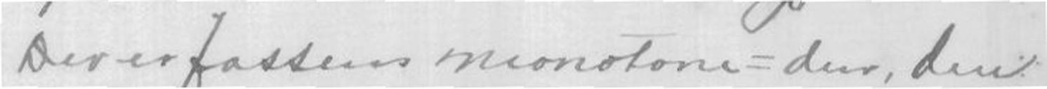Der er fossens monotone=dur, den
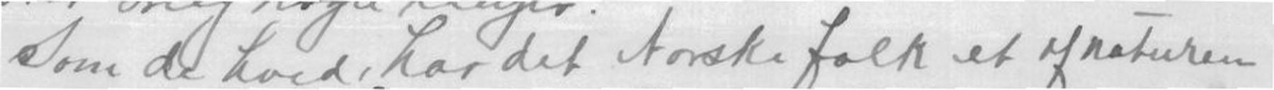Som de hved, har det Norske folk et af naturen
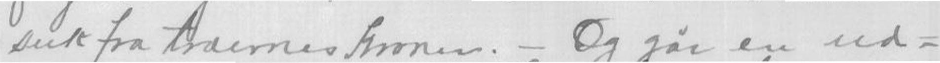suk fra træernes Kroner. - Og går en ud-
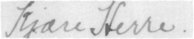Kjære Herre.
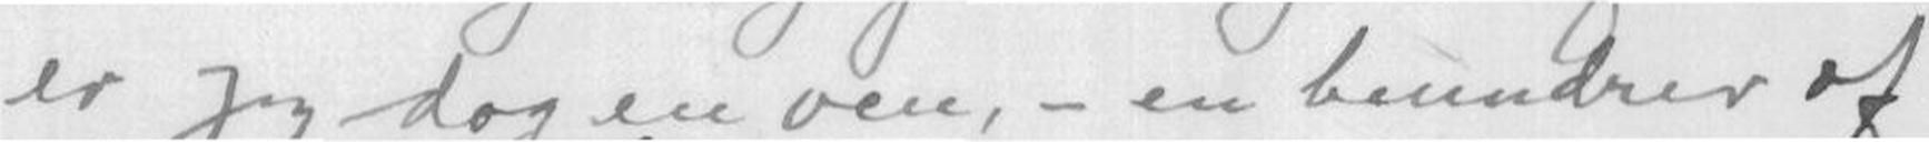er jeg dog en ven, - en beundrer of
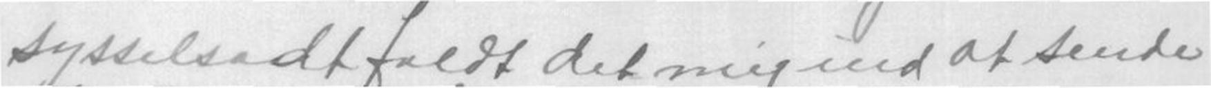sysselsadt faldt det mig ind at sende
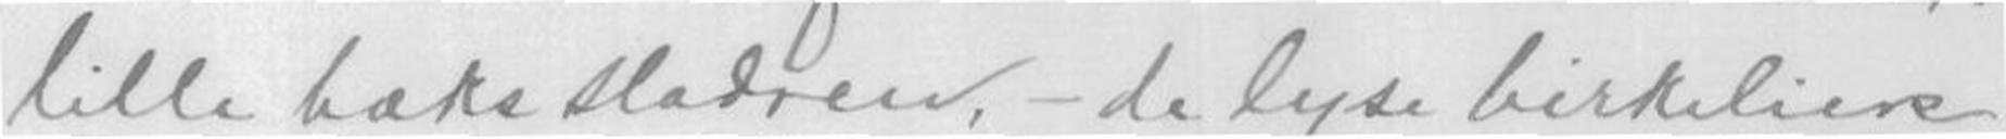lille bæks sladren, - de lyse birkeliers
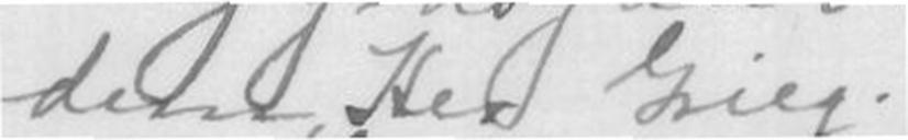dem, Her Grieg.
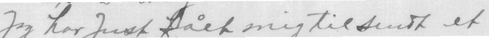Jeg har Just fået mig tilsendt et
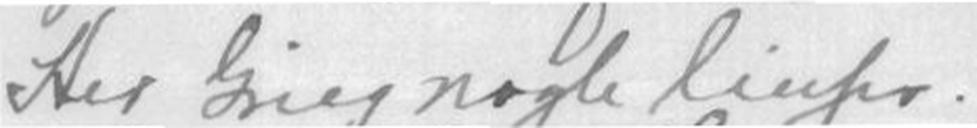Her Grieg nogle linjer.
